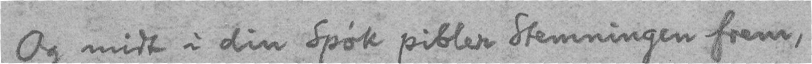Og midt i din Spøk pibler Stemningen frem,
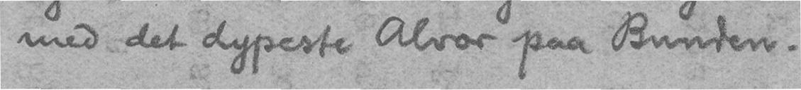med det dypeste Alvor paa Bunden.
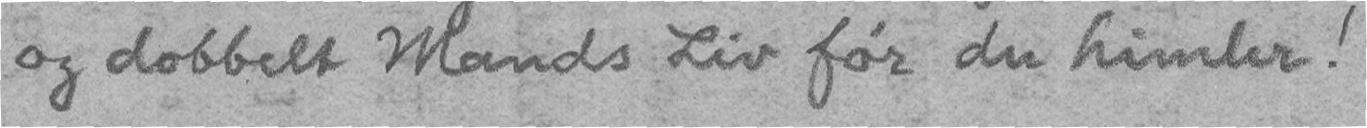og dobbelt Mands Liv før du himler!
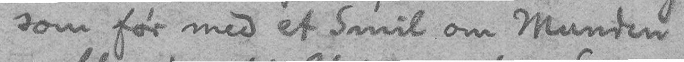som før med et Smil om Munden
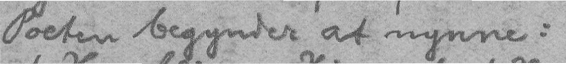Poeten begynder at nynne:
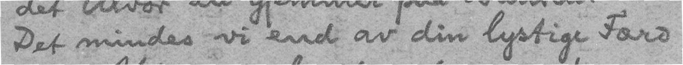Det mindes vi end av din lystige Færd
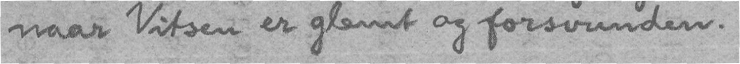naar Vitsen er glemt og forsvunden.
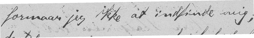formaar jeg ikke at indfinde mig;
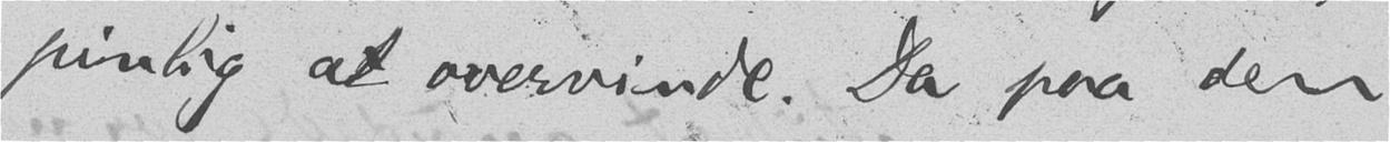pinlig at overvinde. Da paa den
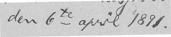den 6te april 1891.
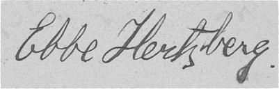Ebbe Hertzberg.
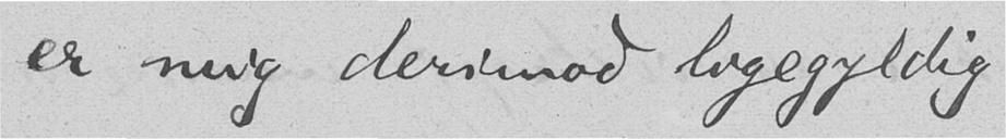er mig derimod ligegyldig.
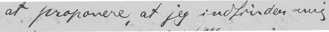at proponere, at jeg indfinder mig
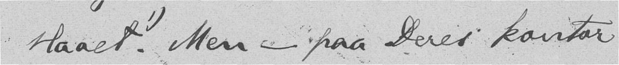slaaet. 1) Men - paa Deres kontor
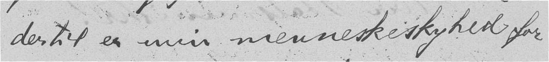dertil er min menneskeskyhed for
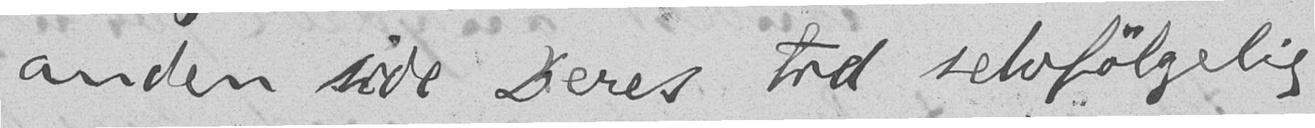anden side Deres tid selvfølgelig
